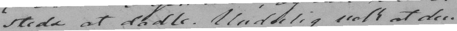steds at dadle. Underlig nok at den
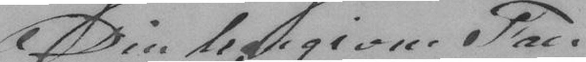Din hengivne Faer
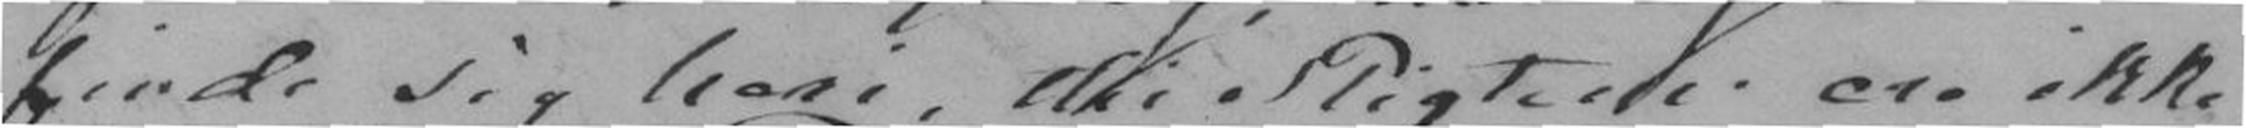finde sig heri, thi Pligten ere ikke
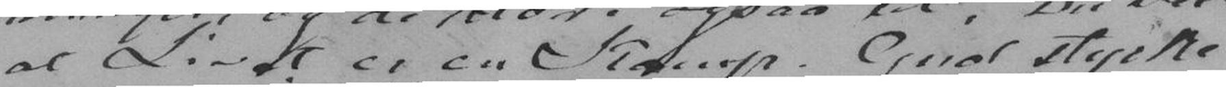at Livet er en Kamp. Gud styrke
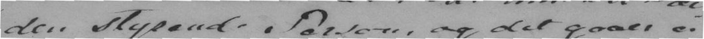den styrende Perosn, og det gaaer ei
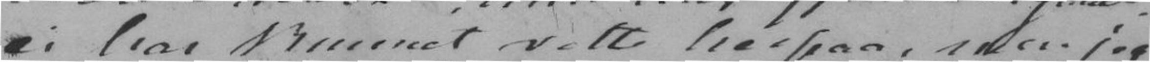ei har kunnet rette herpaa, som jeg
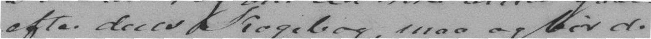efter deres Kogebog, maa og bør de
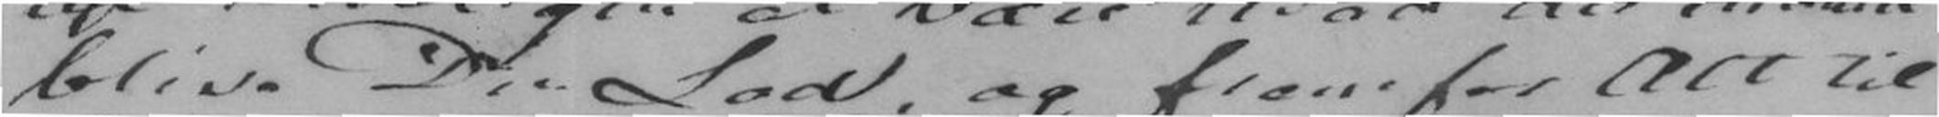blive Din Lod, og fremfor Alt til
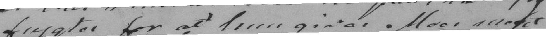frygter for at hun giver Moer meget
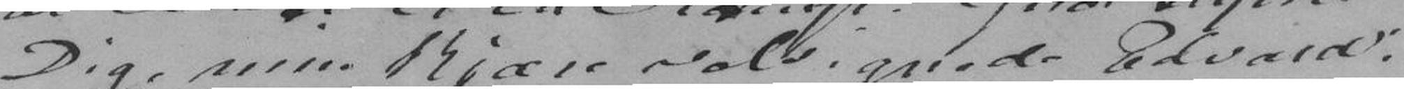Dig, min kjære velsignede Edvard!
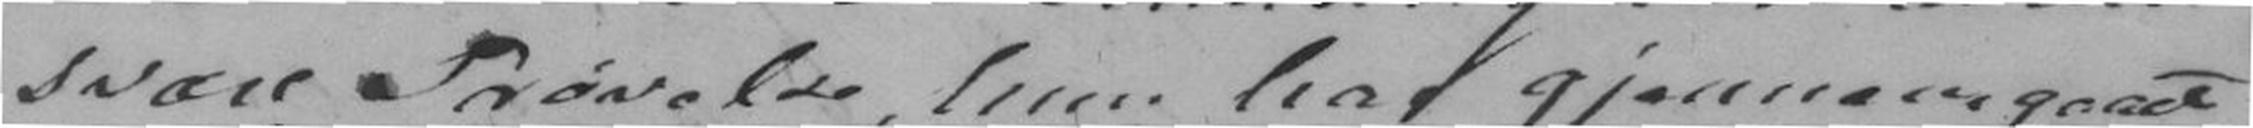svære Prøvelse, hun har gjennemgaaet,
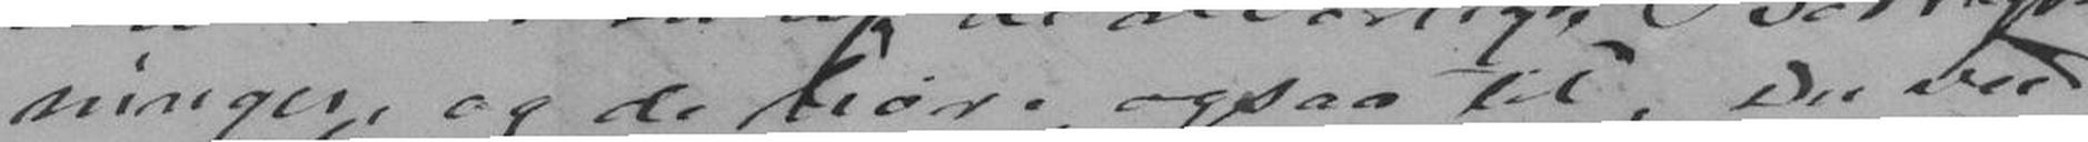ninger, og at høre, ogsaa til, Du veed
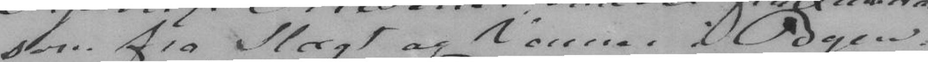som fra Slægt og Venner i Byen,
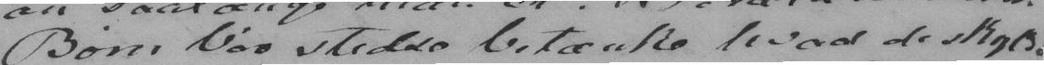Børn bør stedse betænke hvad de skylde
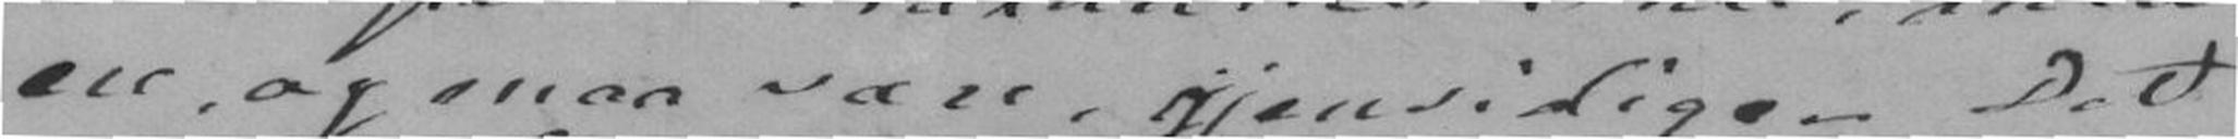ere, og maa være, gjensidige. Det
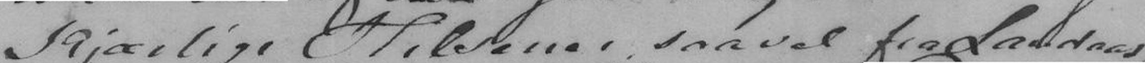Kjærligen Hilsener, saavel fra Landaas
